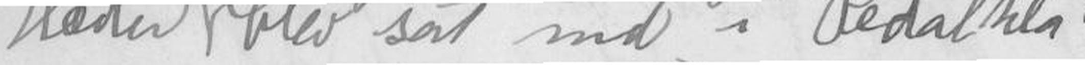Hæder der sad der blev sat ind i Pedalkla-
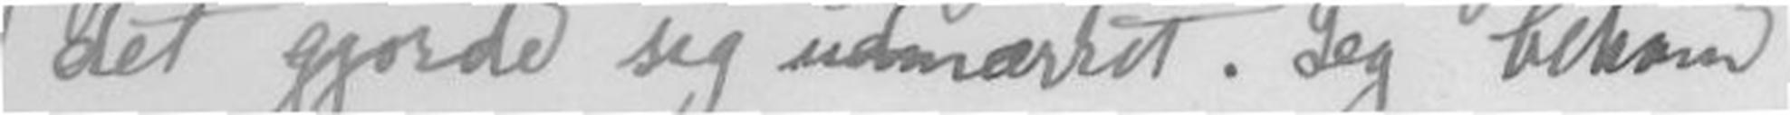det gjorde sig udmærket. Jeg bekom
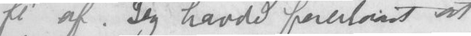fé af. Jeg havde foreslaaet at
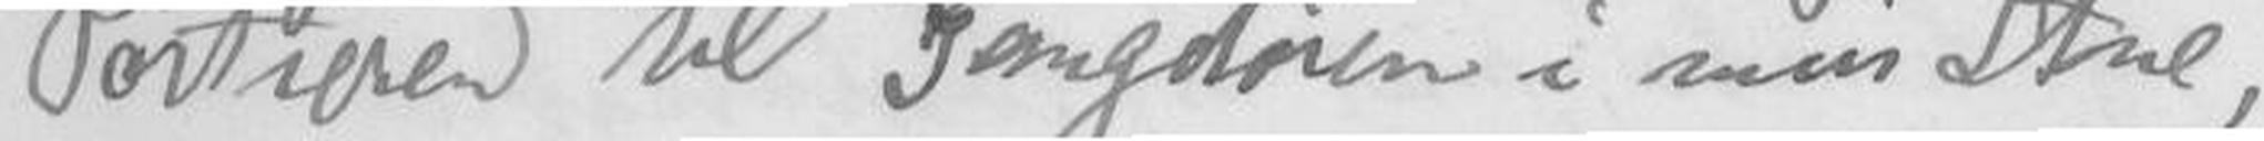Portieren til Gangdøren i min Stue,
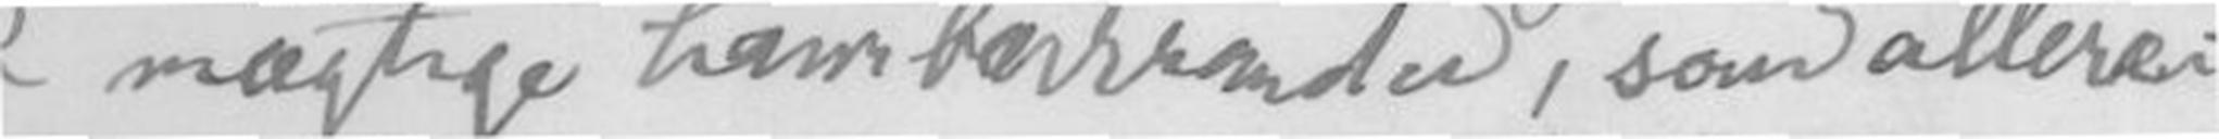2 mægtige Laurbærkrandse, som allere-
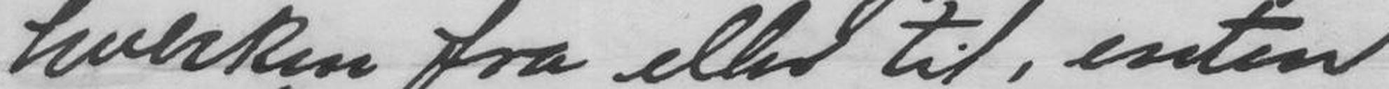hverken fra eller til, enten
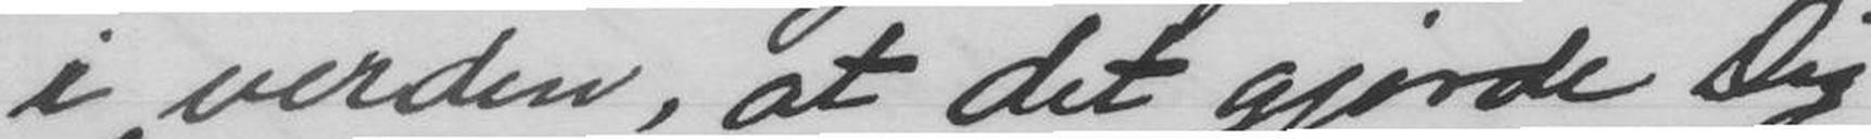i verden, at det gjorde Dig
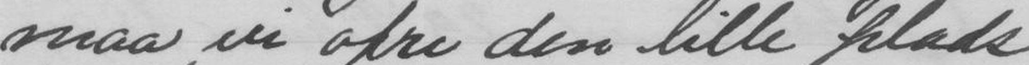maa vi ofre den lille plads
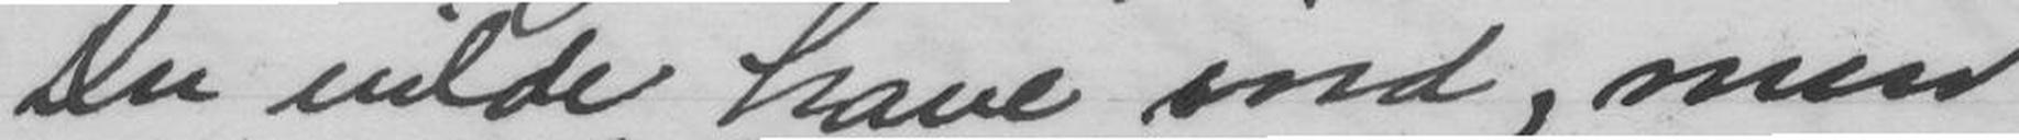Du vilde have ind, men
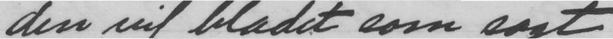den vil bladet som sagt
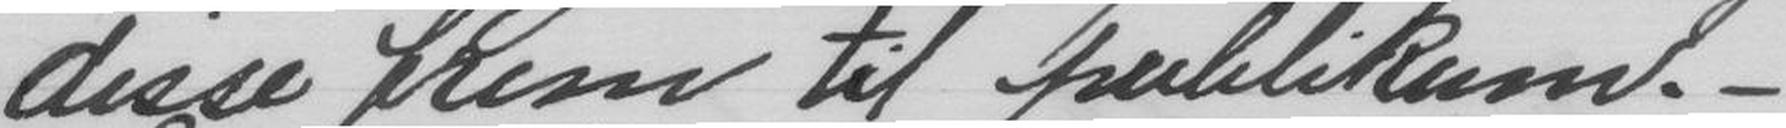disse fem til publikum. -
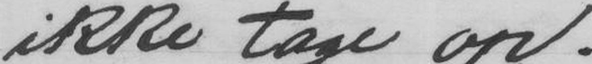ikke tage op.
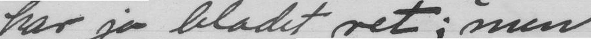har jo bladet ret; men
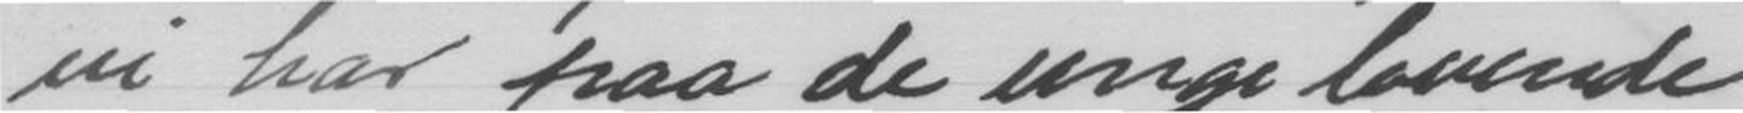vi har paa de unge lovende
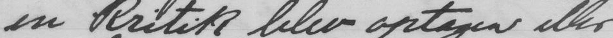en kritik blev optagen eller
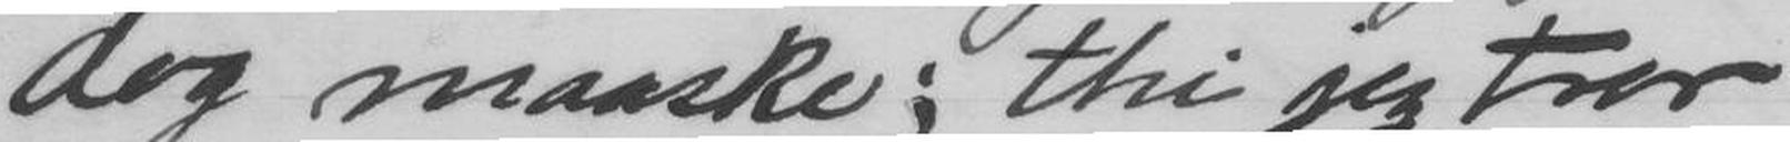dog maaske; thi jeg tror
